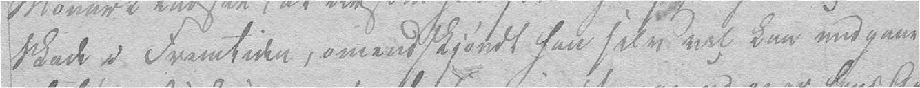Skade i Fremtiden, omendskjøndt han selv vel kan undgaae
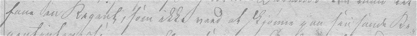faae en Regent, som ikke veed at skjønne paa sin sunde Re-
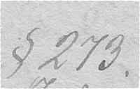§ 273.
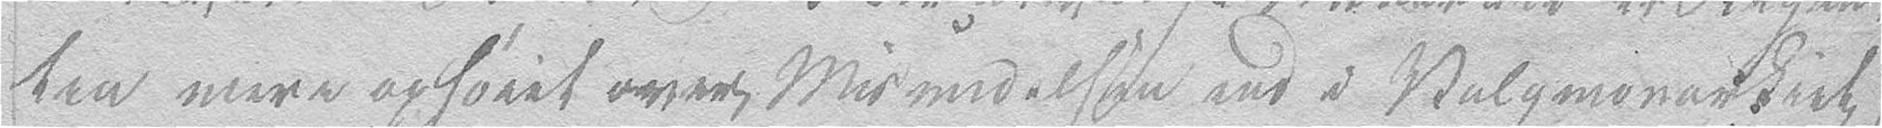ten mere ophøiet over Misundelsen end i Valgmonarkiet,
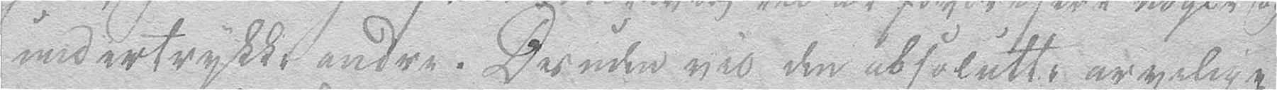undertrykke andre. Desuden vil den absolutte arvelige
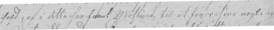hold, og i dette har havt Motiver til at favorisere nogle og
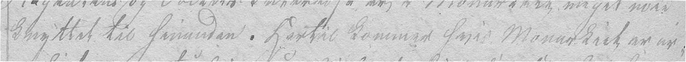knyttet til hinanden. Hertil kommer hvis Monarkiet er ar-
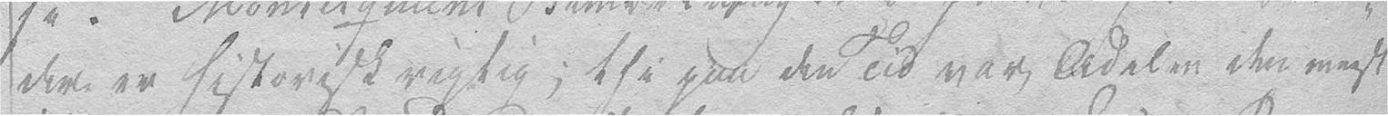der er historisk rigtig; thi paa den Tid var Adelen den meest
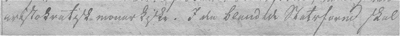aristokratisk-monarkiske. I den blandede Statsform skal
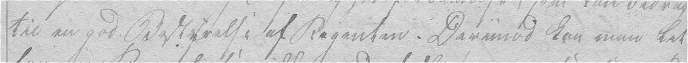til en god Bestyrelse af Regenten. Derimod kan man let
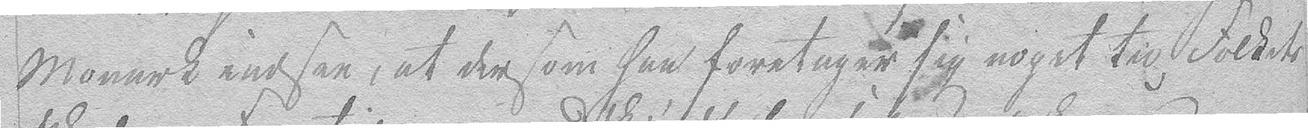Monark indsee, at dersom han foretager sig noget til Folkets
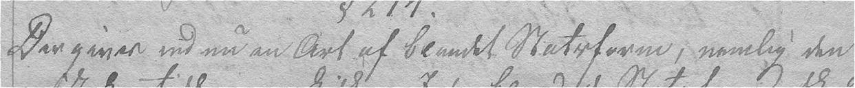Der gives end nu en Art af blandet Statsform, nemlig den
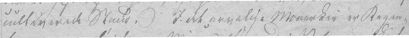cultiverede Stand. I det arvelige Monarkie er Regen-
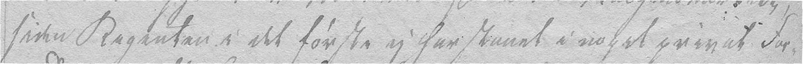siden Regenten i det første ey har staaet i noget privat For-
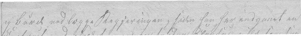ey burde nedlægge Regjeringen, siden han har indgaaet en
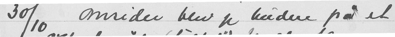30/10 Omsider blev jeg buden på et
